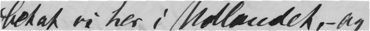betat os her i Udlandet, - og
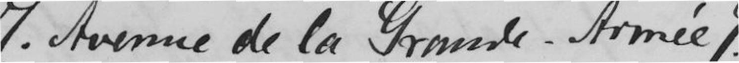7. Avenue de la Grande - Armée 7.
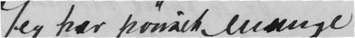Jeg har prøvet mange
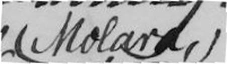Molard, -
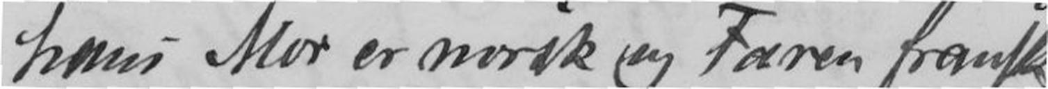hans Mor er norsk og Faren fransk.
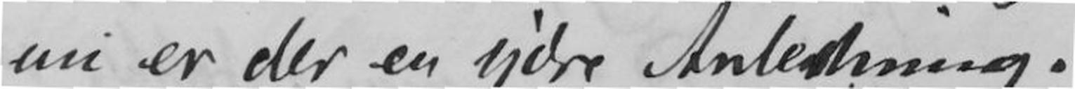nu er der en ydre Anledning.
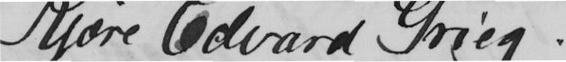Kjære Edvard Grieg!
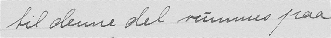til denne del rummes paa
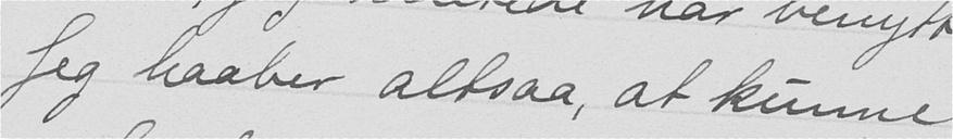Jeg haaber altsaa, at kunne
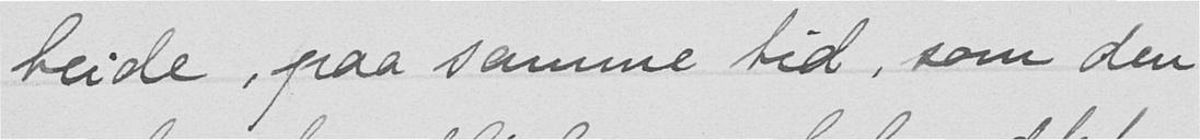beide, paa samme tid, som den
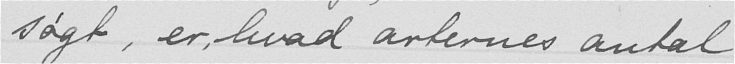søgt, er hvad arternes antal
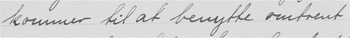kommer til at benytte omtrent
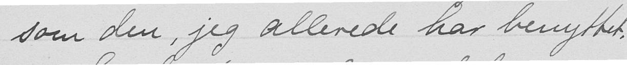som den, jeg allerede har benyttet.
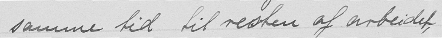samme tid til resten af arbeidet
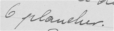6 plancher.
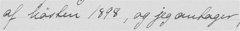af høsten 1898, og jeg antager
